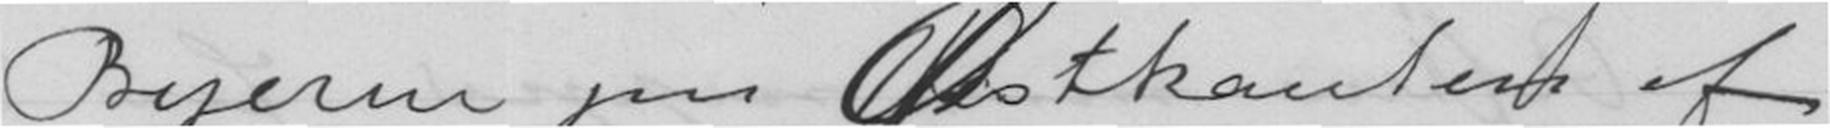Byerne paa Østkanten af
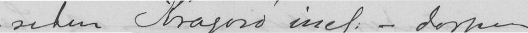siden Kragerø incf: - derpaa
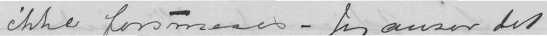ikke forsmaaes - Jeg anser det
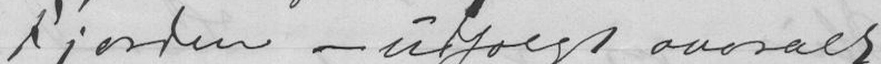Fjorden - udsolgt overalt
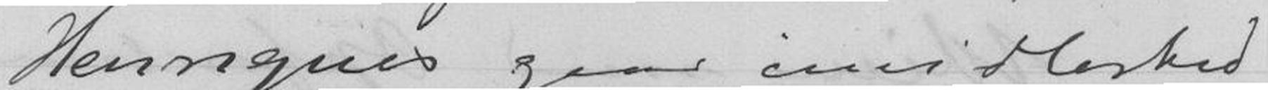Henriques gaar imidlertid
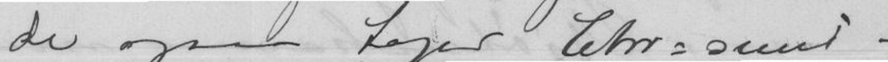de ogsaa tager Chr=sund
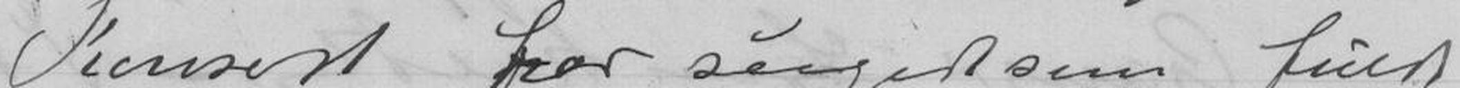Konsert stor saagodt som fuldt
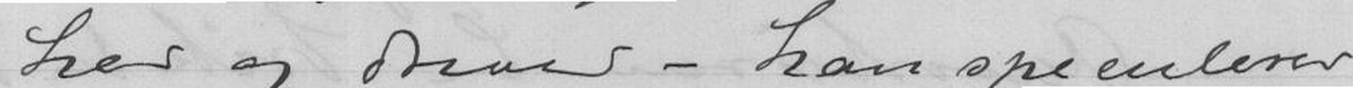her og driver - han speculerer
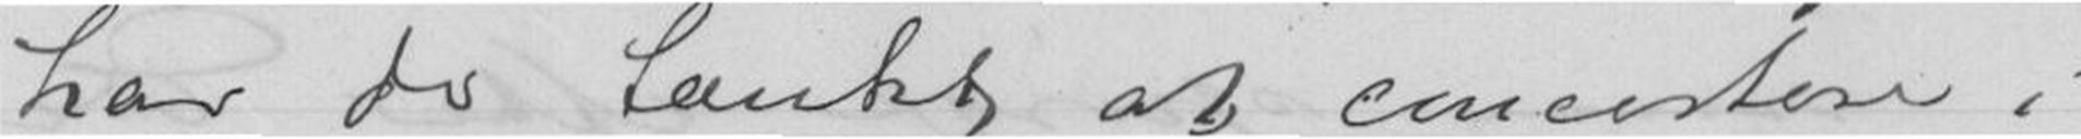har de tænkt at comcertere i
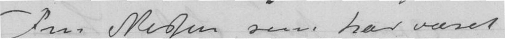Fru Nissen som har været
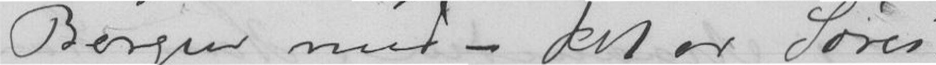Bergen med - det er Sørei
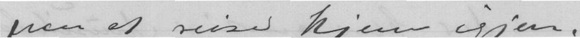paa at reise hjem igjen.
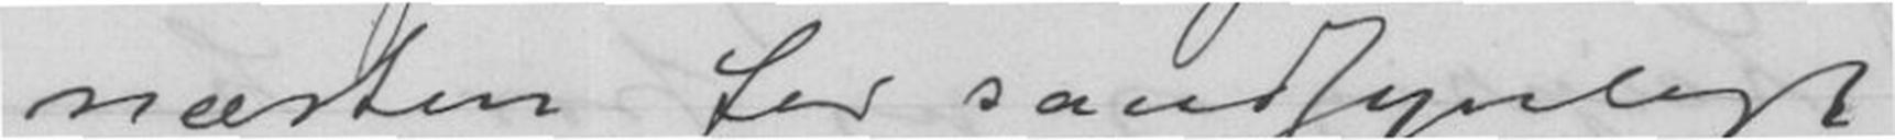næsten for sandsynligt
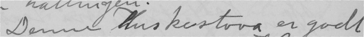Denne Huskestova er godt
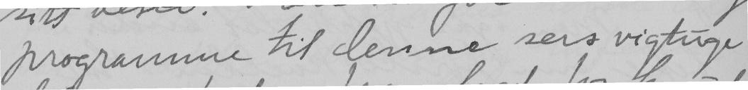programme til denne sers vigtige
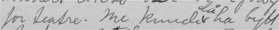for teatre. Me kunde då ha bytt
